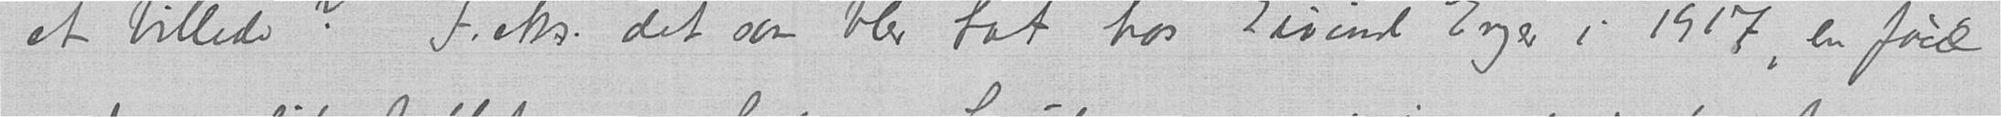et billede? F.eks. det som blev tat hos Eivind Enger i 1917, en frice
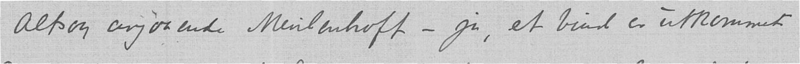Altsaa angaaende Meulenhoff - ja, et bind er utkommet
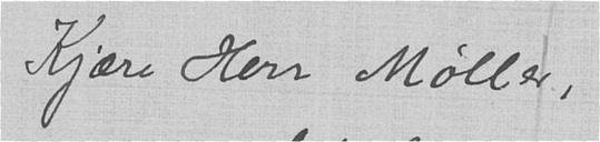Kjære Herr Møller,
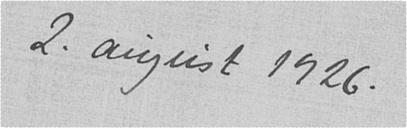2. august 1926.
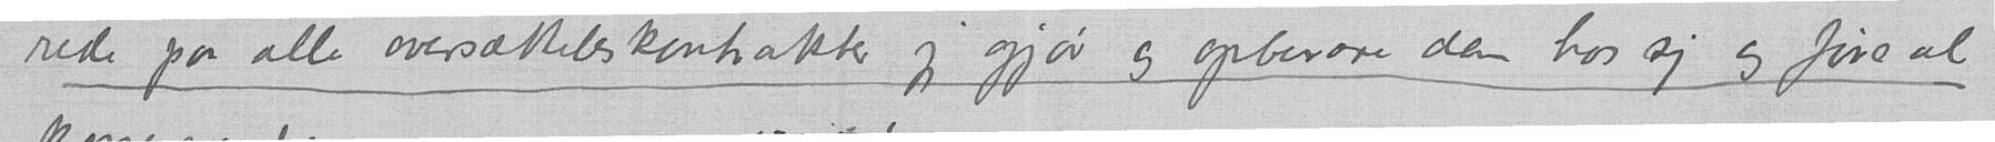rede paa alle oversætteleskontrakter jeg gjør og opbevare dem hos sig og føre al
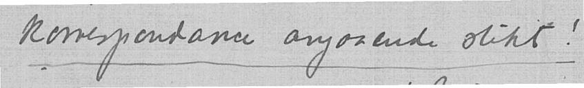korrespondance angaaende slikt!
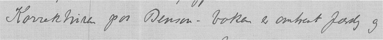Korrekturen paa Benson-boken er omtrent færdig og
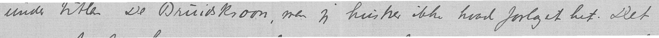under titlen De Druidskroon, men jeg husker ikke hvad forlaget het. Det
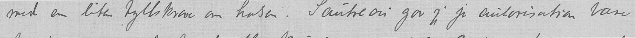med en liten tyllskrave om halsen. Sautreau gav jeg jo autorisation bare
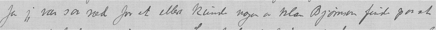for jeg var saa ræd for at ellers kunde nogen av klan Bjørnson finde paa at
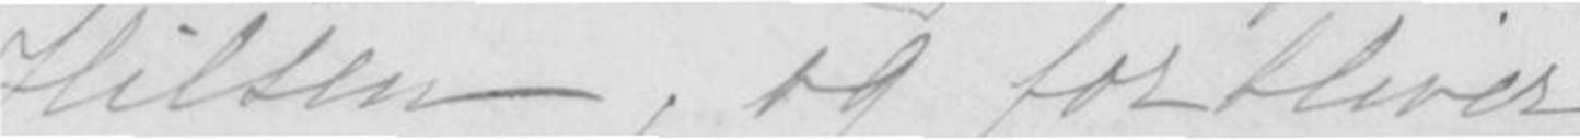Hilsen og forbliver
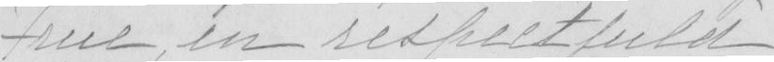Frue, en respectfuld
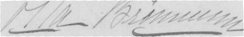Otta Brönnum
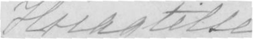Høiagtelse
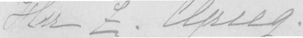Hrr E. Grieg.
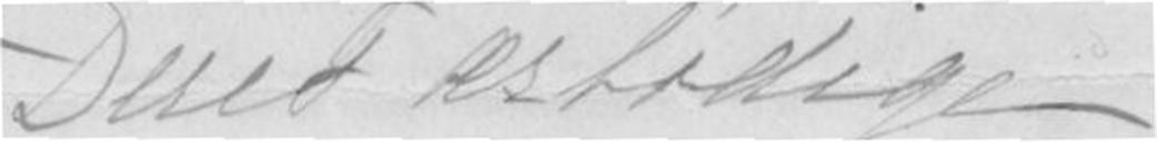Deres ærbødige
